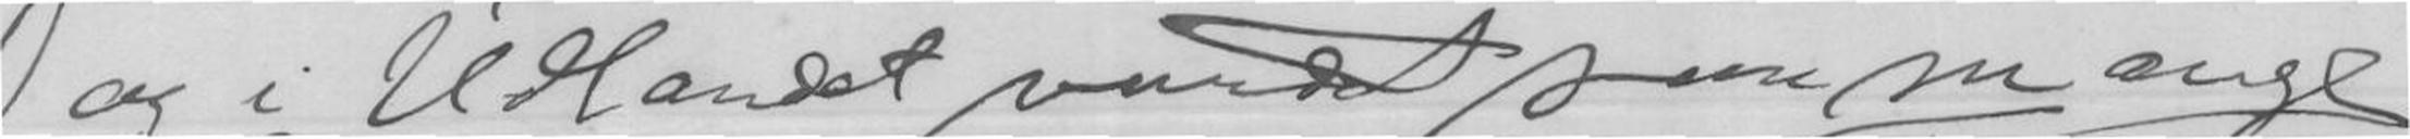og i Udlandet vundet saa mange
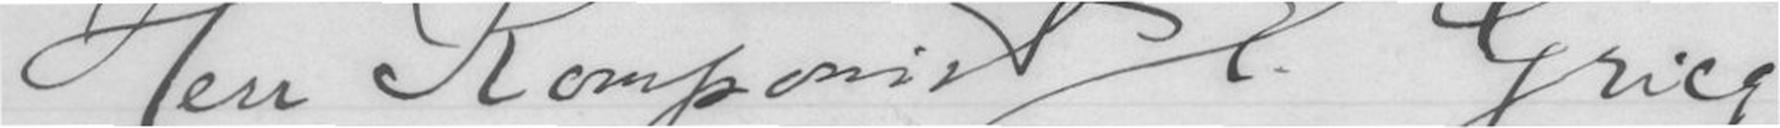Herr Komponist E. Grieg!
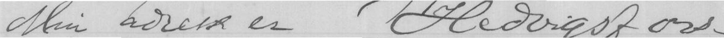Min adresse er Hedvigsfors
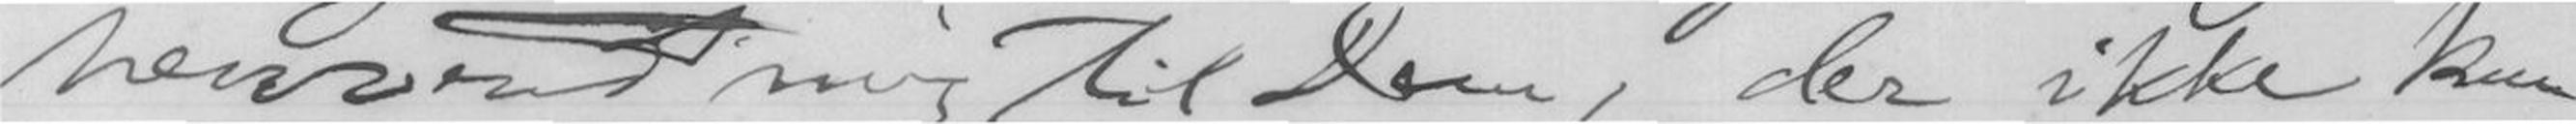henvender mig til Dem, der ikke kun
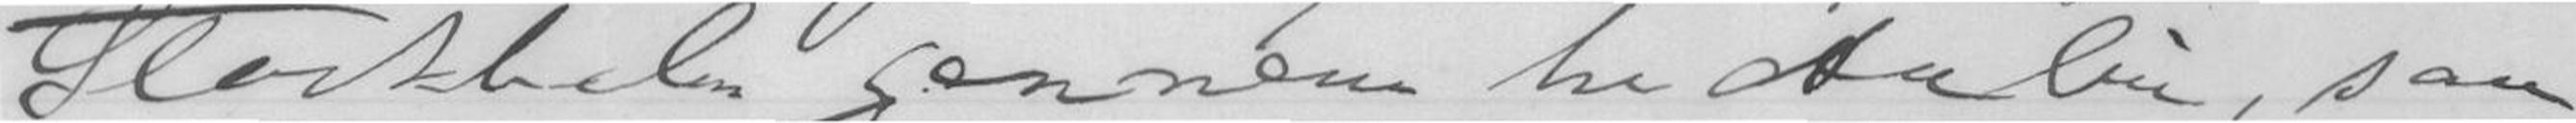Stockholm gennem her Aulin, saa
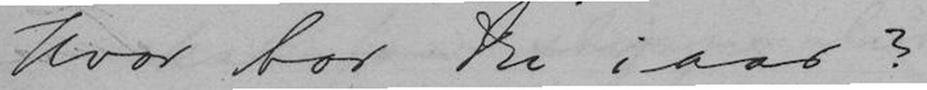Hvor bor Du iaar?
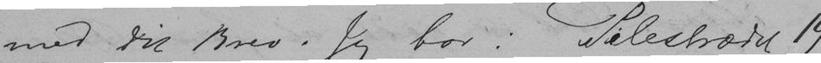med Dit Brev. Jeg bor i Pilestrædet 19.
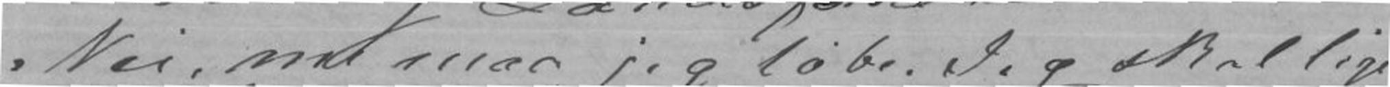Nei, nu maa jeg løbe. Jeg skal lige
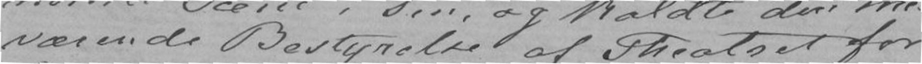værende Bestyrelse af Theatret for
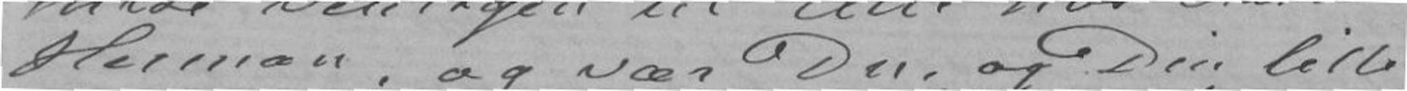Herman, og vær Du og Din lille
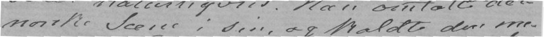norske Scene i sin, og koldte den nu-
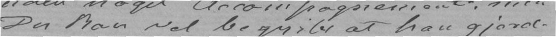Du kan vel begribe at han gjorde
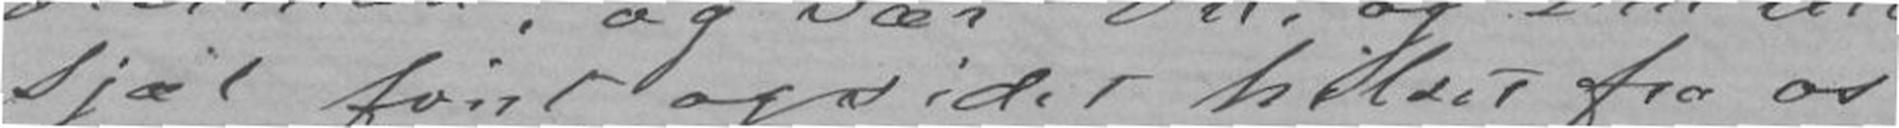sjæl først og sidst hilset fra os
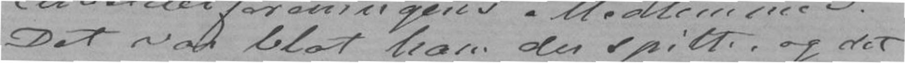Det var blot ham der spilte, og det
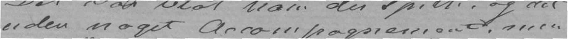uden noget Accompognement, men
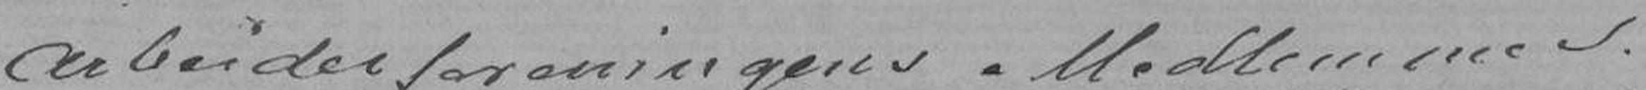Arbeiderforeningens Medlemmer.
